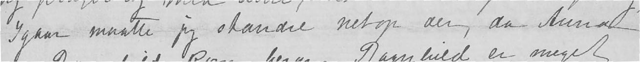Igaar maatte jeg standse netop der, da Anna
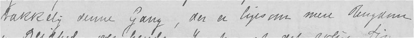tækkelig denne Gang, der er ligesom mere Ungdom
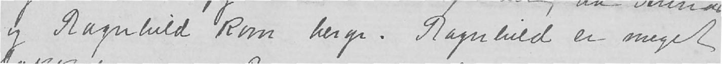og Ragnhild kom herop. Ragnhild er meget
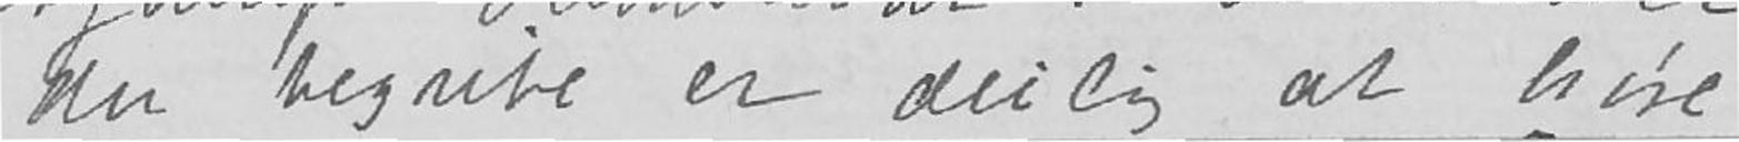du begribe er deilig at høre.
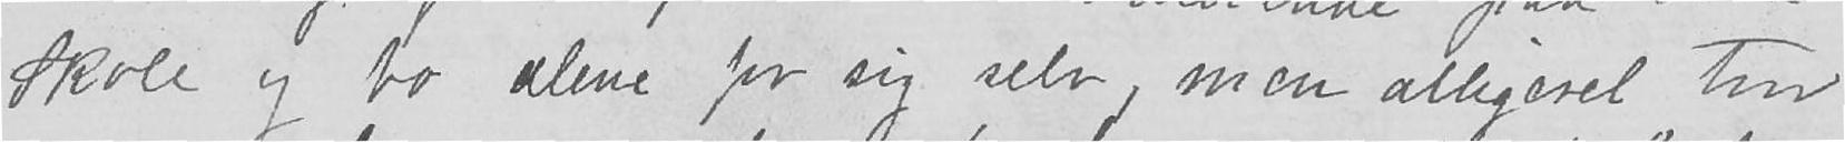Skole og bo alene for sig selv, men alligevel tror
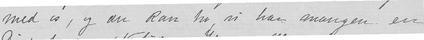med os, og du kan tro, vi har mangen en
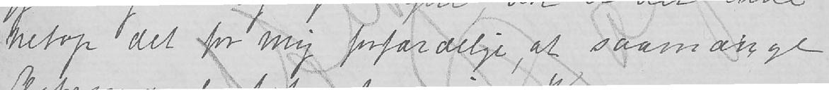netop det for mig forfærdelige, at saamange
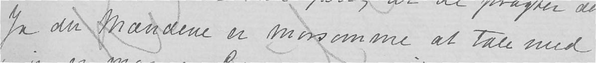Ja du Mændene er morsomme at tale med
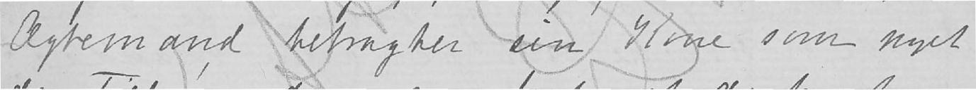Ægtemænd betragter sin Kone som noget
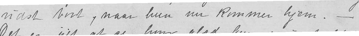vidst bort, naar hun nu kommer hjem. –
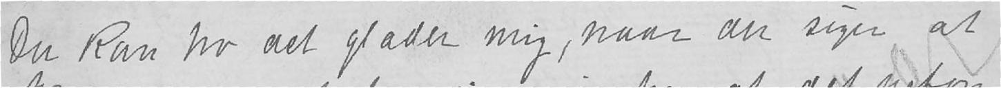Du kan tro det glæder mig, naar du siger at
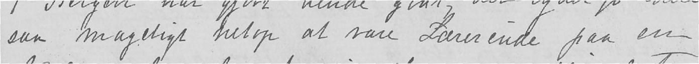saa mageligt netop at være Lærerinde paa en
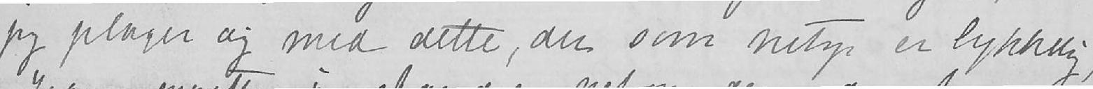jeg plager dig med dette, du som netop er lykkelig,
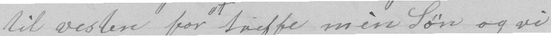til vesten for at treffe min Søn og vi
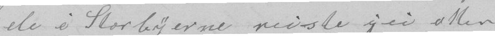de i Storbyerne reiste jei atter
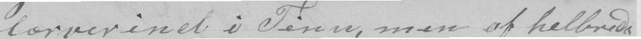lærrerind i Tinn, men af helbreds
